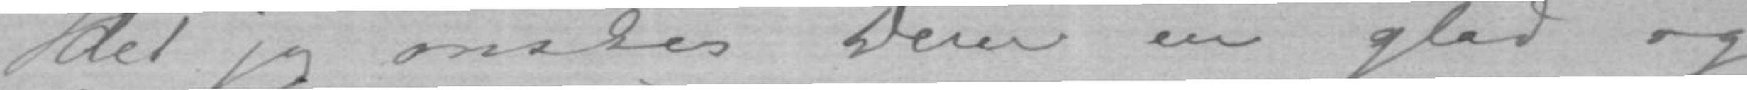Idet jeg ønsker Dem en glad og
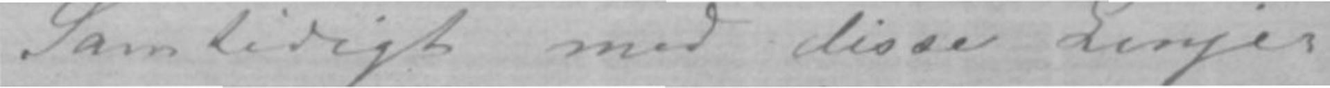Samtidigt med disse Linjer
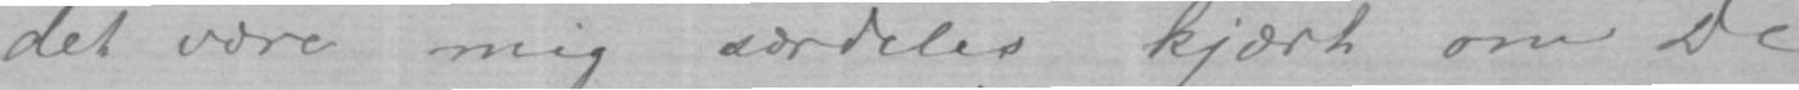det være mig særdeles kjært om De
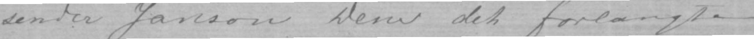sender Janson Dem det forlangte
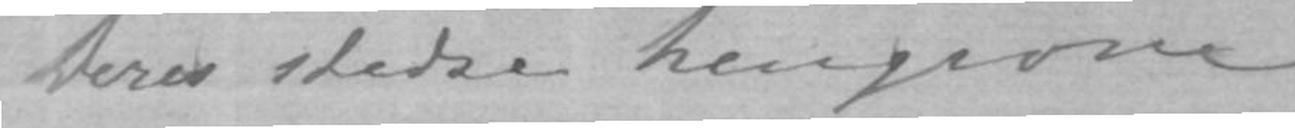Deres stedse hengivne
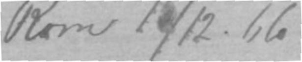Rom 15/12. 66.
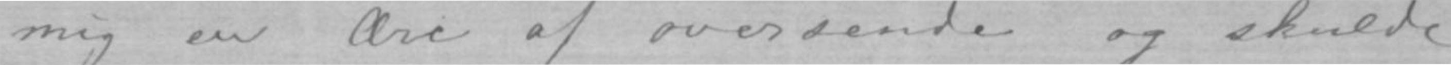mig en Ære af oversende og skulde
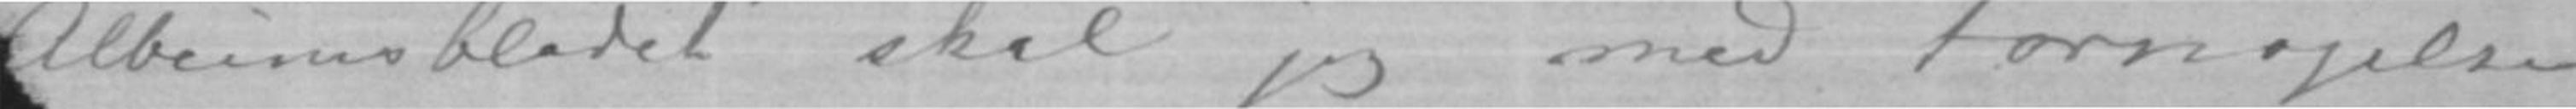Albumsbladet skal jeg med Fornøjelse
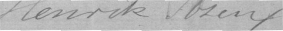Henrik Ibsen.
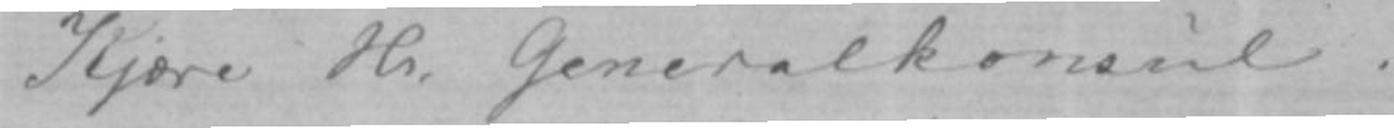Kjære Hr. Generalkonsul!
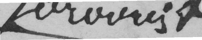forøvrigt
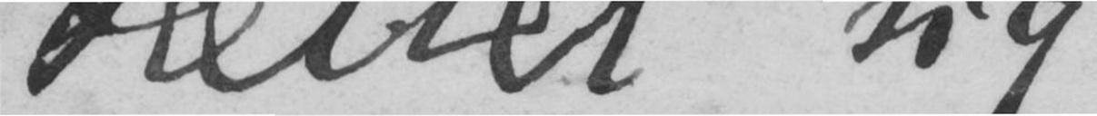tættet sig
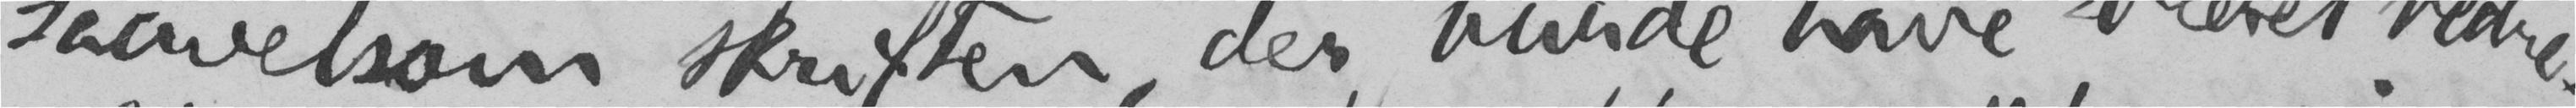saavelsom skriften, der burde have været bedre.
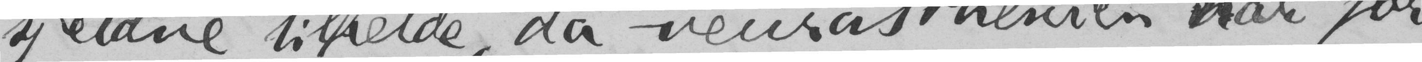sjeldne tilfælde, da neurasthenien har for-
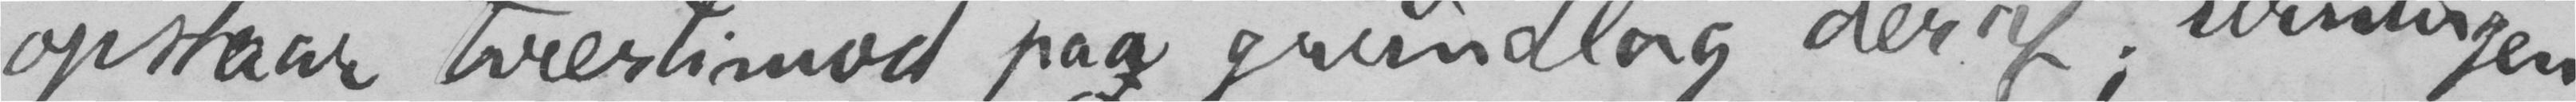opstaar tværtimod paa grundlag deraf; urningen
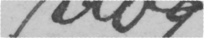dog
